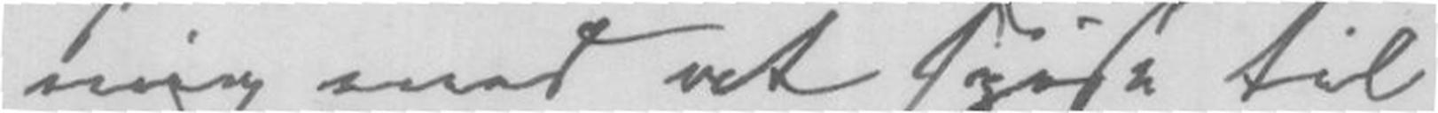mig ved at spise til
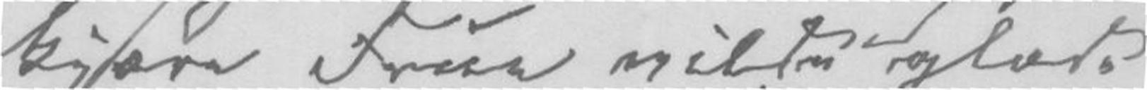kjære Frue vilde glæde
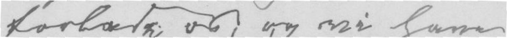forlade os, og vi have
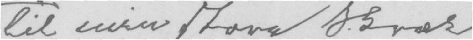Til min store Skræk
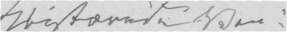Højstærede Ven!
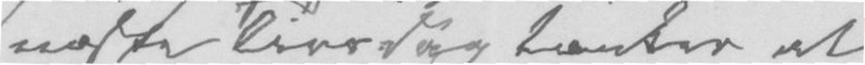næste Tirsdag tænker at
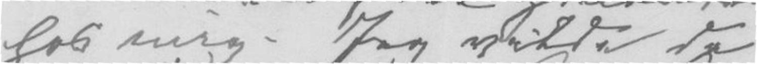hos mig. Jeg vilde da
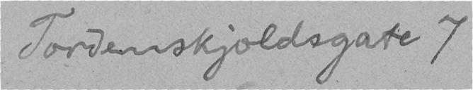Tordenskjoldsgate 7
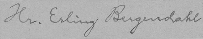Hr. Erling Bergendahl
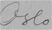Oslo
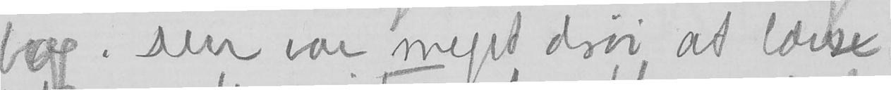bog. Den var meget drøi at læse
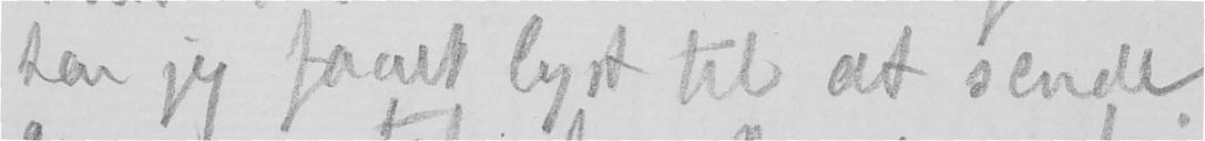har jeg faaet lyst til at sende
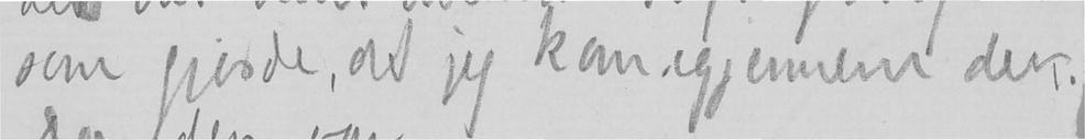som gjorde, at jeg kom igjennem den.
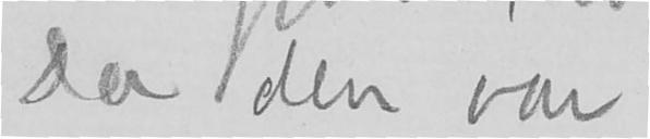Da den var
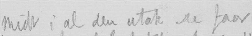Midt i al den utak De faar
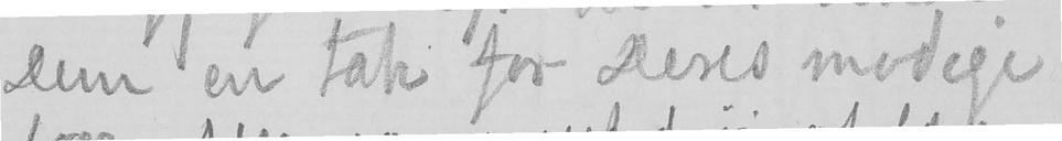Dem en tak for Deres modige
